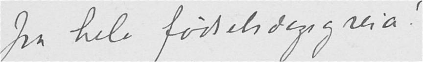fra hele fødselsdagsgreia!
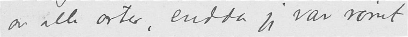av alle arter, endda jeg var rømt
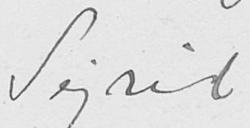Sigrid.
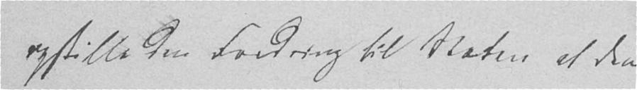opstille den Fordring til Staten at den
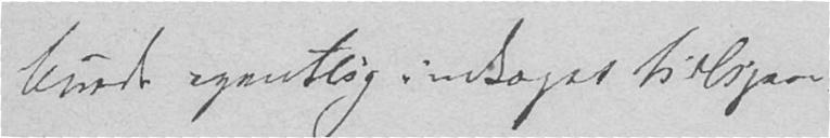Burde egentlig indtages tidligere.
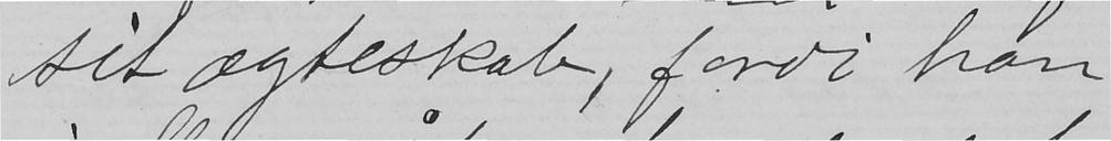sit ægteskab, fordi han
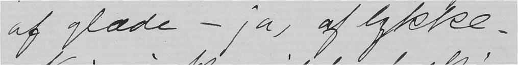af glæde - ja, af lykke.
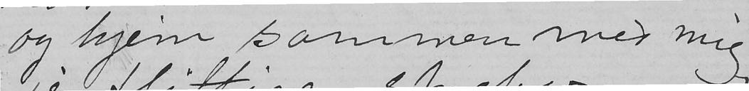og hjem sammen med mig,
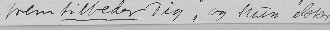frem tilbeder Dig, og hun elsker
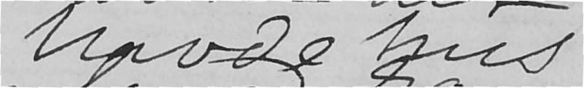havde hus
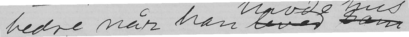bedre når han leved sam
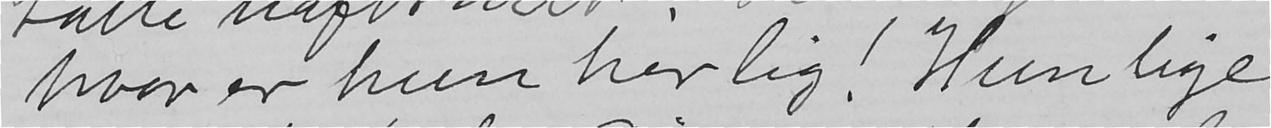hvor er hun herlig! Hun lige
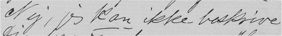Nej, jeg kan ikke beskrive
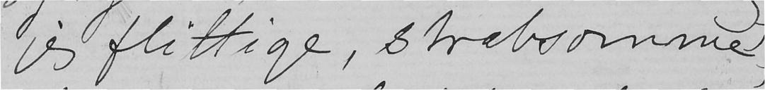jeg flittige, stræbsomme,
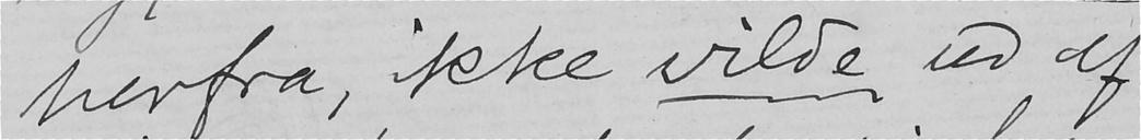herfra, ikke vilde ud af
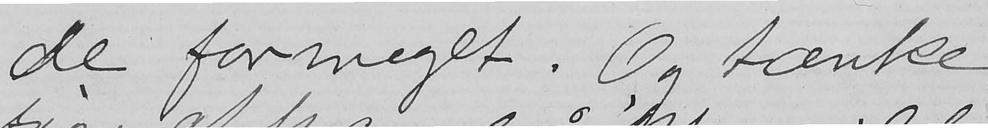de formeget. O tænke
